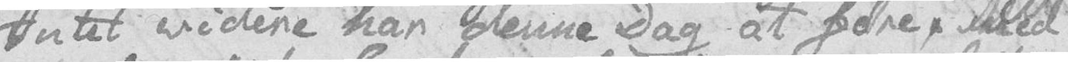Intet videre har denne Dag at føre. Med
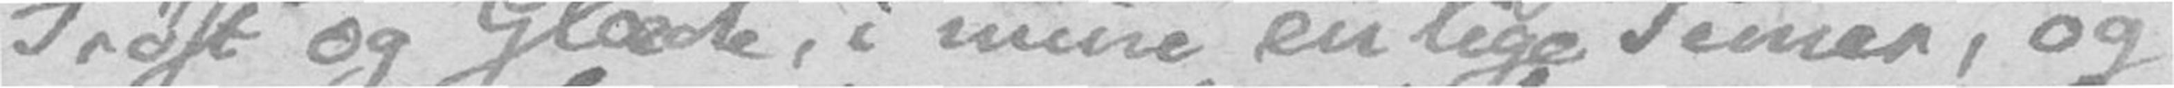Trøst og Glæde, i mine enlige Timer, og
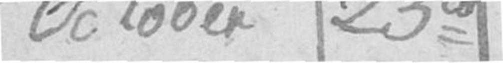October 23de
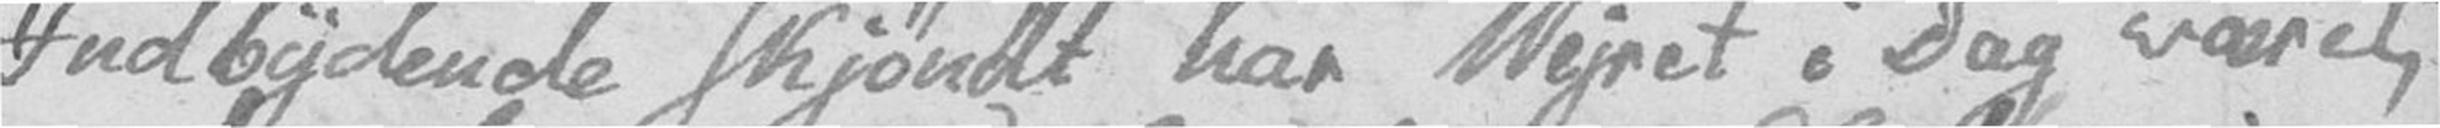Indbydende skjøndt har Vejret i Dag været,
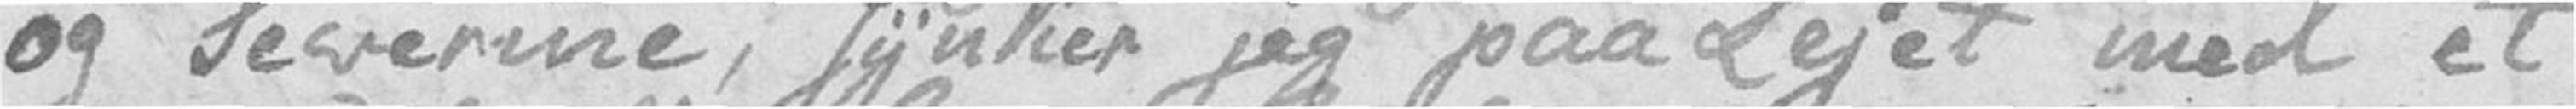og Severine, synker jeg paa Lejet med et
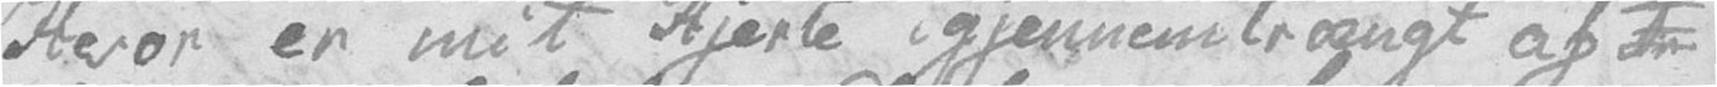Hvor er mit Hjerte igjennemtrængt af
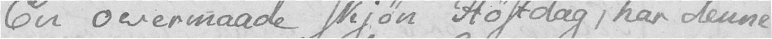En overmaade skjøn Høstdag, har denne
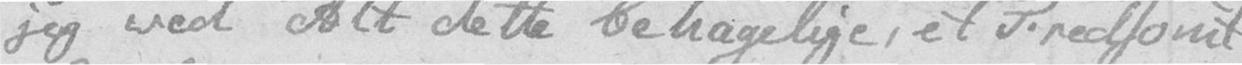jeg ved Alt dette behagelige, et Fredsomt
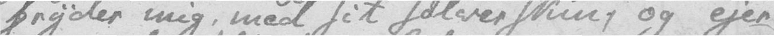fryder mig, med sit sølverskin, og ejer
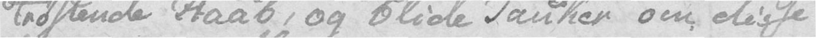trøstende Haab, og blide Tanker om disse
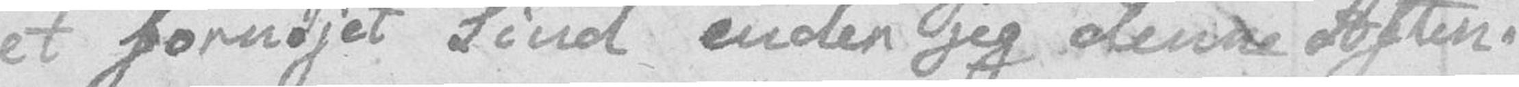et fornøjet Sind ender jeg denne Aften.
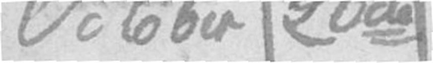October 20de
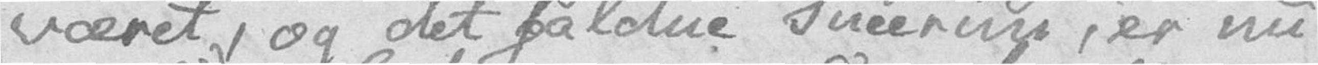været, og det faldne Sneerim, er nu
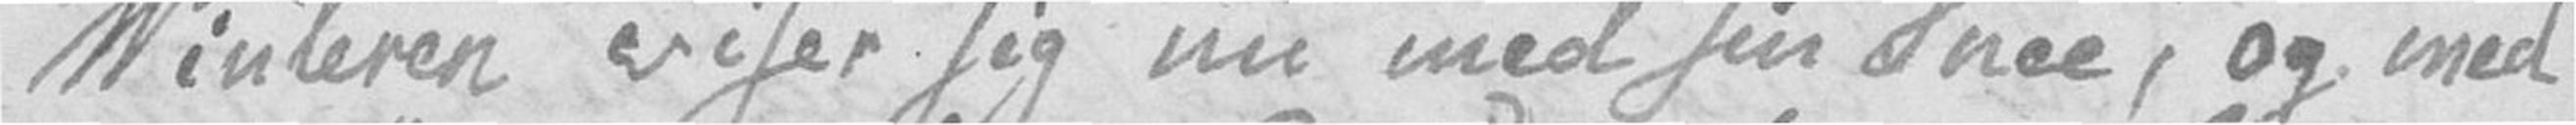Vinteren viser sig nu med sin Snee, og med
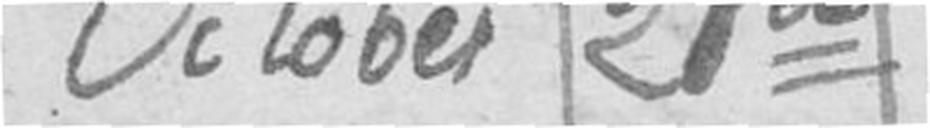October 21de
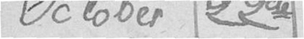October 22de
